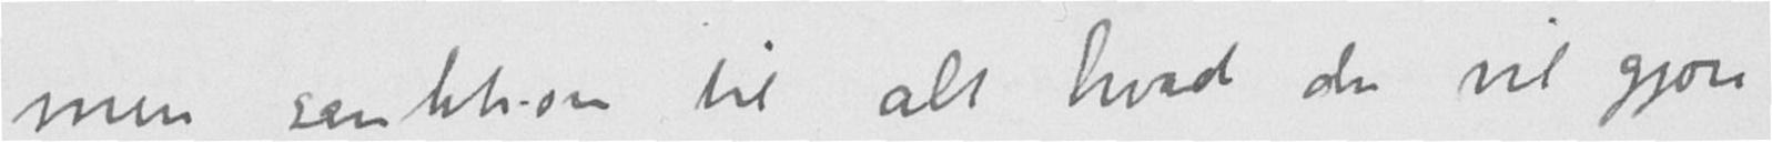min sanktion til alt hvad du vil gjøre
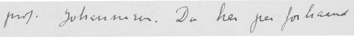prof. Johannesen. Du har paa forhaand
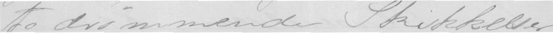to drømmende Skikkelser
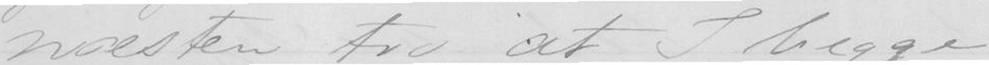næsten tro at I begge
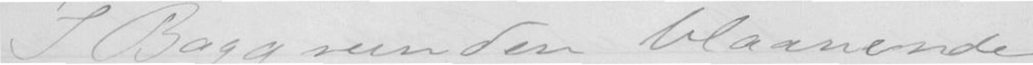I Baggrundens blaanende
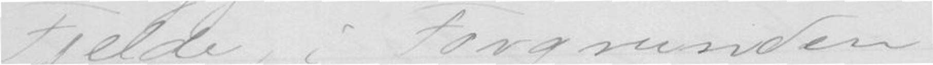Fjelde, i Forgrunden
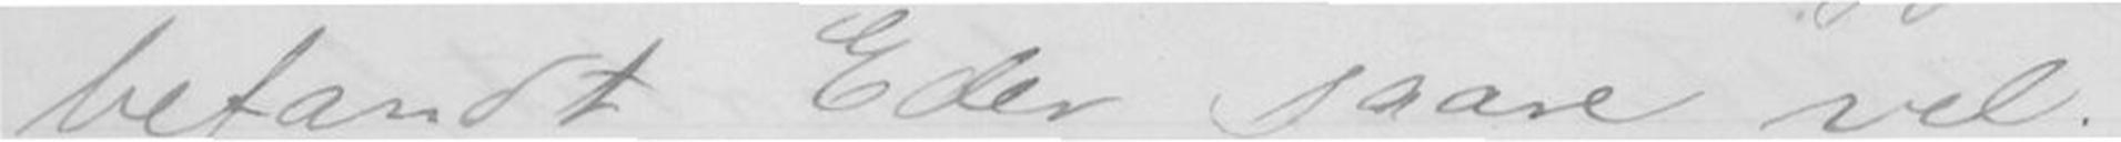befandt Eder saare vel.
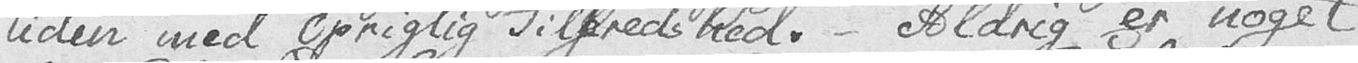tiden med oprigtig Tilfredshed. – Aldrig er noget
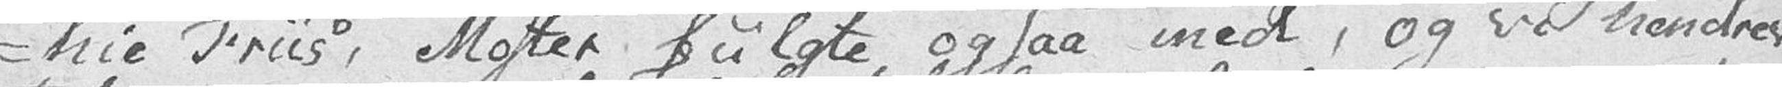-hie Friis, Moster fulgte ogsaa med, og vi hendrev
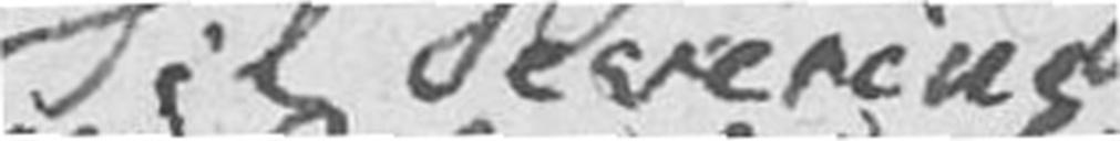Til Severine
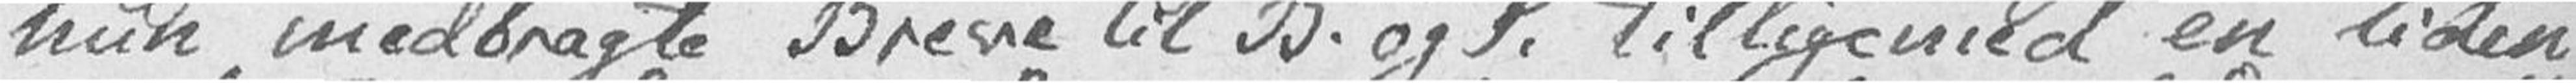hun medbragte Breve til B. og S. tilligemed en liden
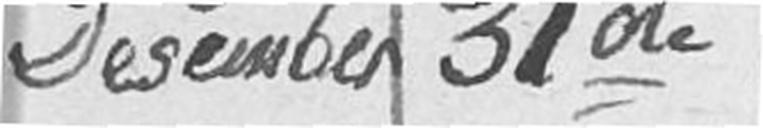Desember 31de
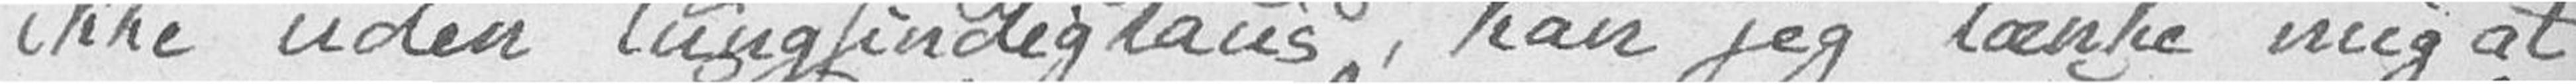ikke uden tungsindig taus, kan jeg tænke mig at
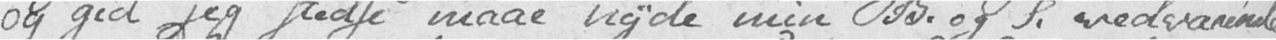og gid jeg stedse maae nyde min B. og S. vedvarende
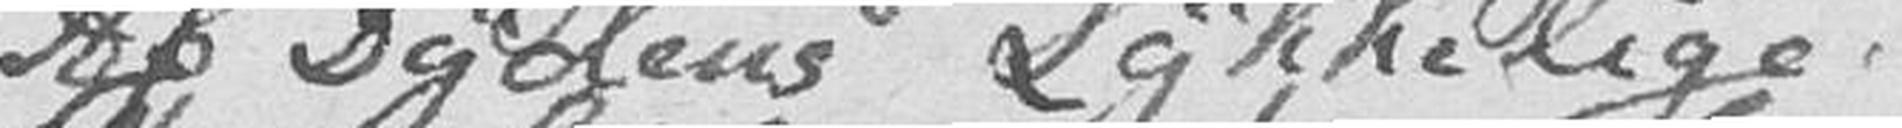Af Dydens Lykkelige,
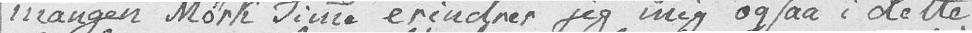mangen Mørk Timme erindrer jeg mig ogsaa i dette
